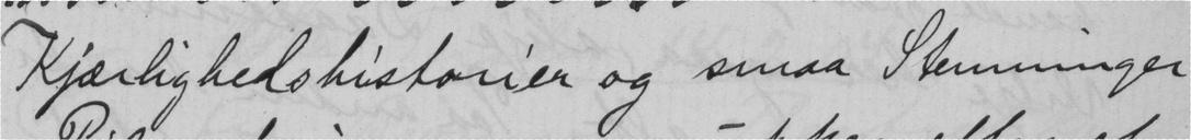Kjærlighedshistorier og smaa Stemninger
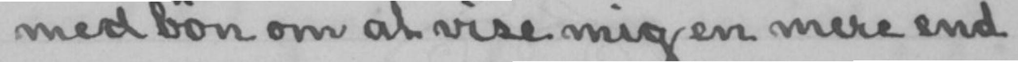med bøn om at vise mig en mere end
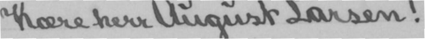Kære herr August Larsen!
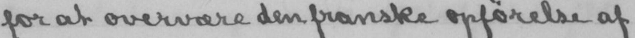for at overvære den franske opførelsen af
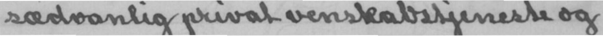sædvanlig privat venskabstjeneste og
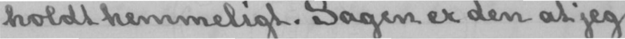holdt hemmeligt. Sagen er den at jeg
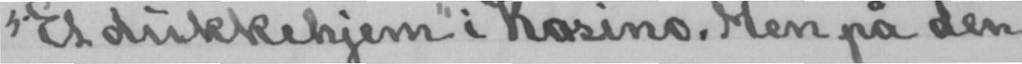«Et dukkehjem» i Kasino. Men på den
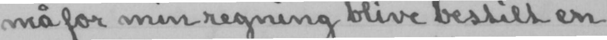må for min regning blive bestilt en
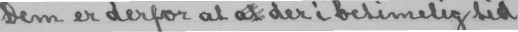Dem er derfor at at der i betimelig tid
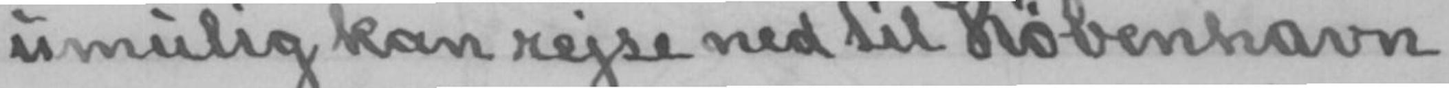umulig kan rejse ned til København
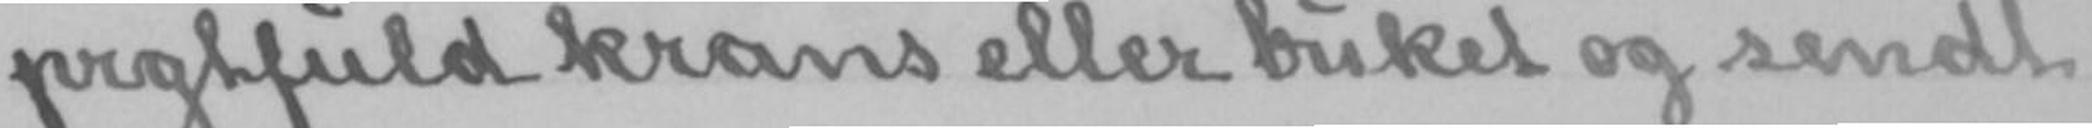prgtfuld krans eller buket og sendt
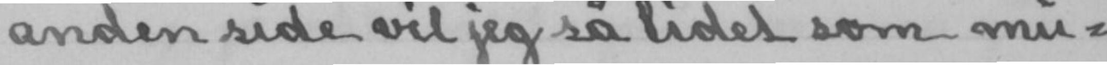anden side vil jeg så lidet som mu-
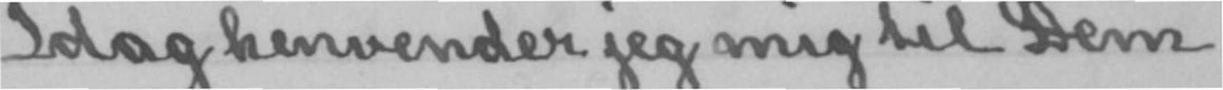Idag henvender jeg mig til Dem
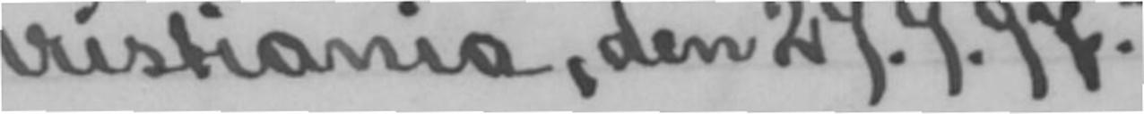Kristiania, den 29.9.97.
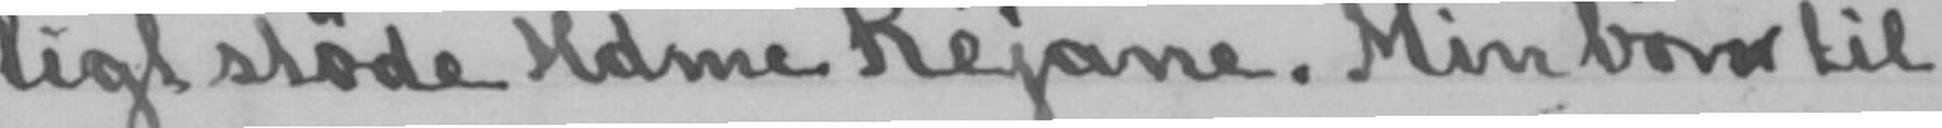ligt støde Mdme Réjane. Min bøn til
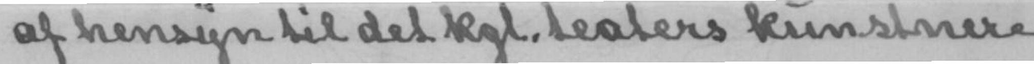af hensyn til det kgl. teaters kunstnere
